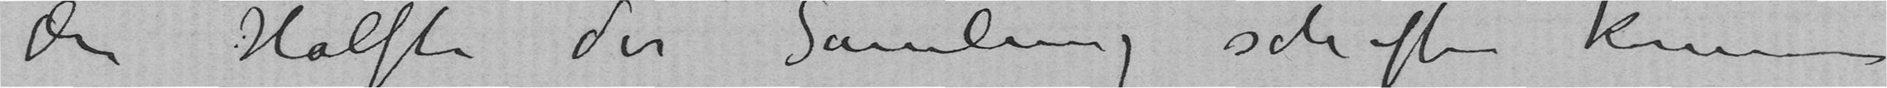der Halfte der Samlung schaften konnen
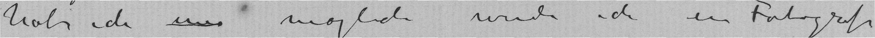habe ich m moglich werde ich ein Fotografi
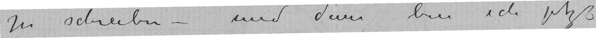zu schreiben – und denn bin ich jetzt
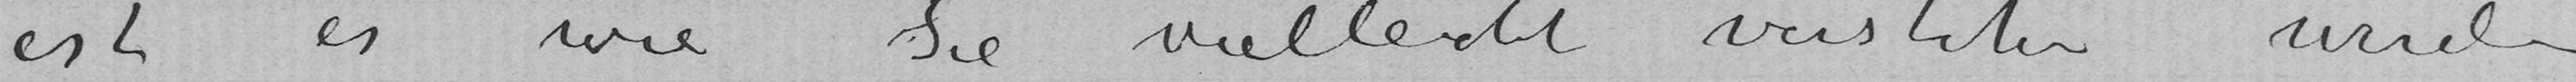ist es wie Sie vielleicht verstehe wieder
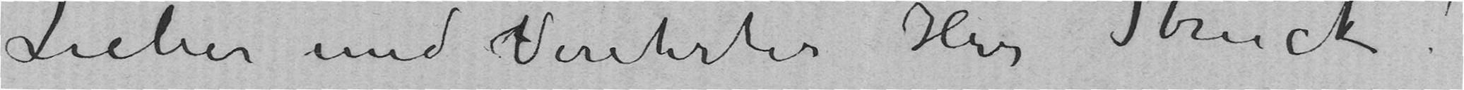Lieber und Verehrter Hrr Struck!
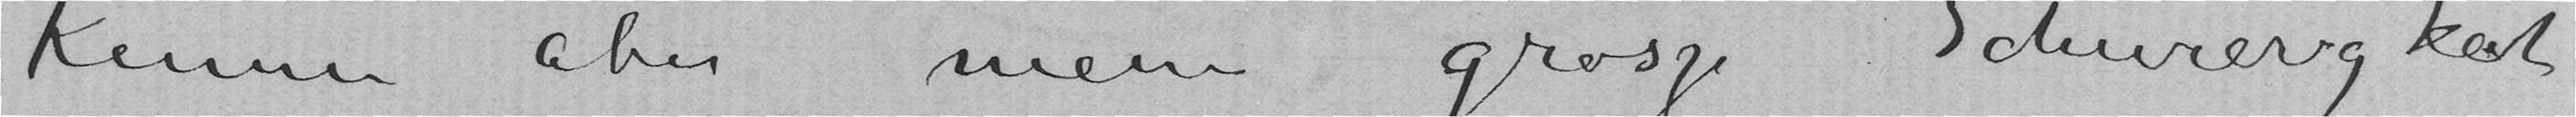kennen aber mein grosze Schwierigkeit
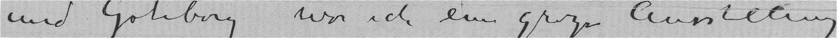und Goteborg wo ich ein grosze Ausstellung
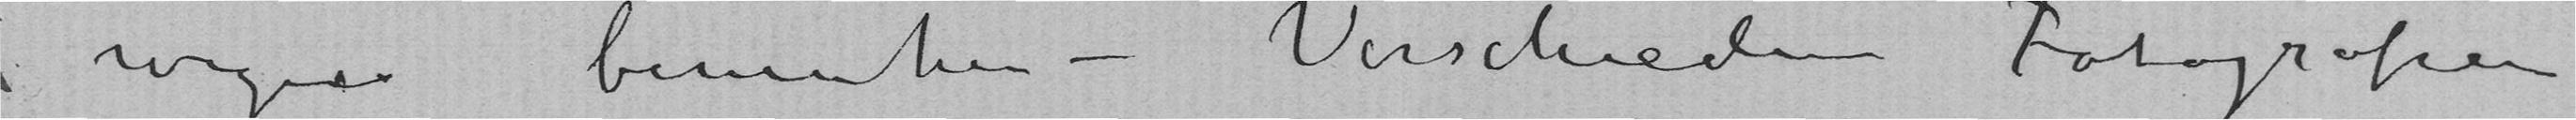wegen bemuhen – Verschieden Fotografien
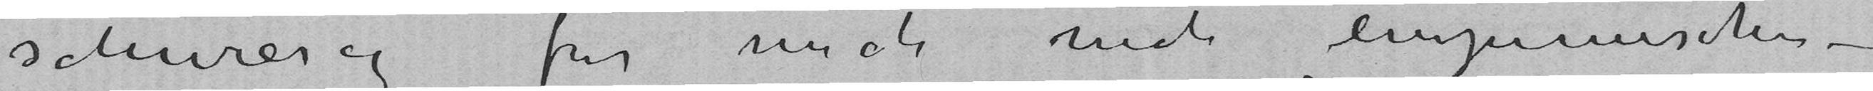schwierig fur mich noch einzumischen –
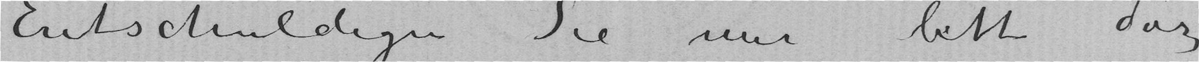Entschuldigen Sie mir bitte dasz
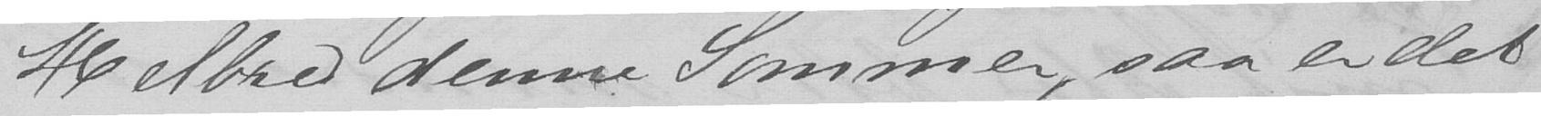Helbred denne Sommer, saa er det
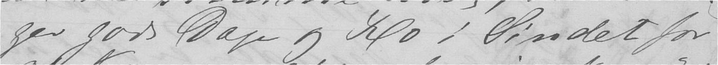ger gode Dage og Ro i Sindet for
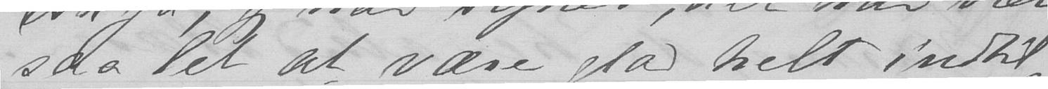saa let at være glad helt indtil
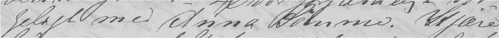geligt med Anna Sommer. Kjære,
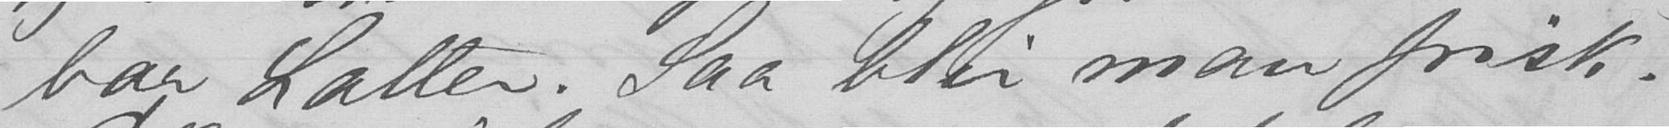bar Latter. Saa blir man frisk.
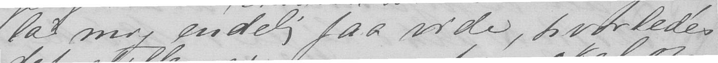lad mig endelig faa vide, hvorledes
